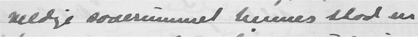veldige soverummet hennes stod en
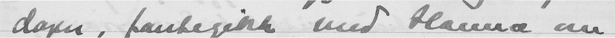dagen, fantegikk med Hanna om
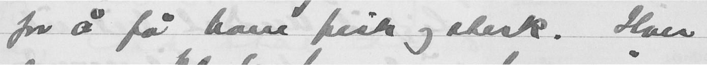for å få ham frisk og sterk. Hver
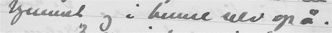hjemmet og i henne selv også.
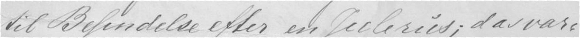til Befindelse efter en Julereis; desvær-
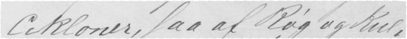Cekloner, faa af Røg og Kul
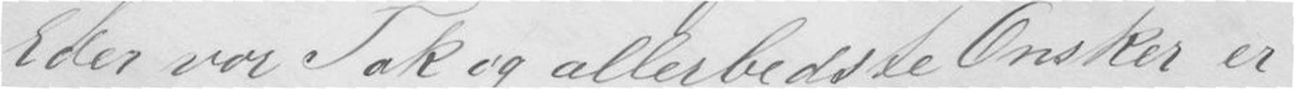Eder vor Tak allerbedste Ønsker er
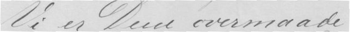Vi er Dere overmaade
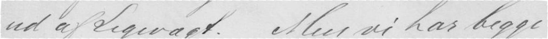ud af Ligevægt. Men vi har begge
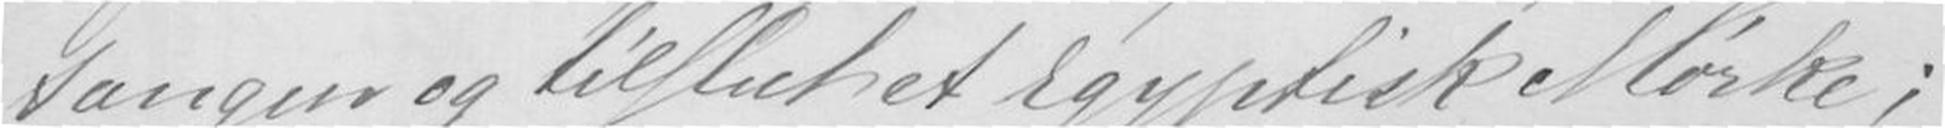sangen og tilflukt et Egyptisk Mørke;
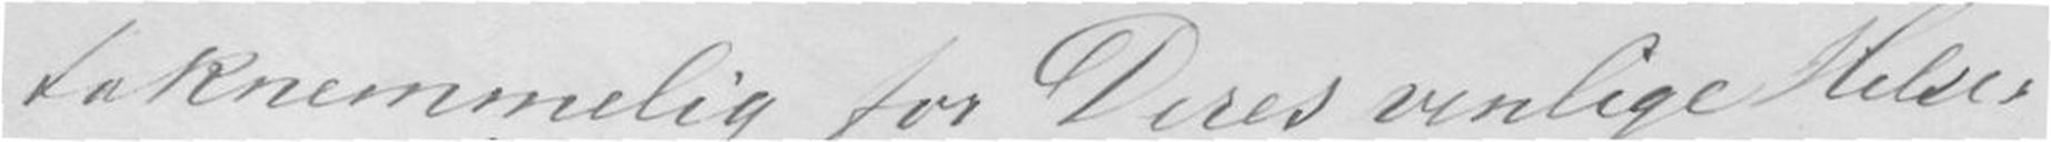taknemmelig for Deres venlige Hilse-
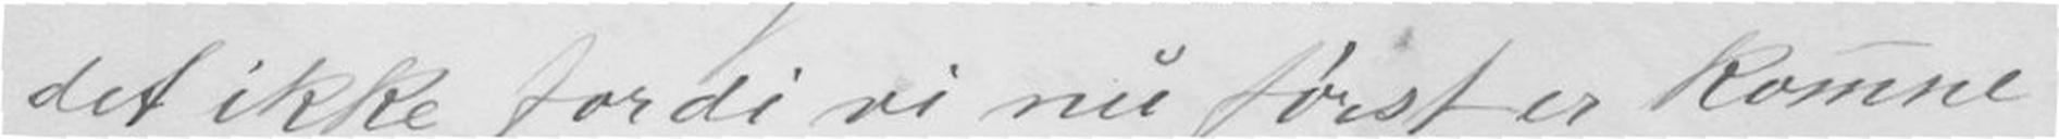det ikke fordi vi nu først er komne
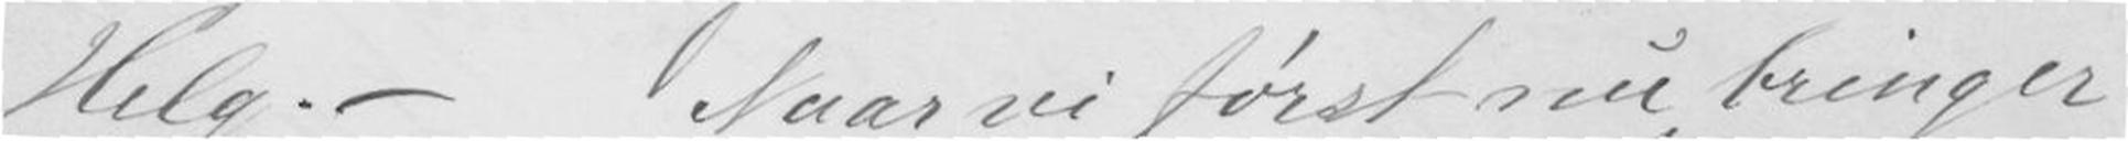Helg.- Naar vi først nu bringer
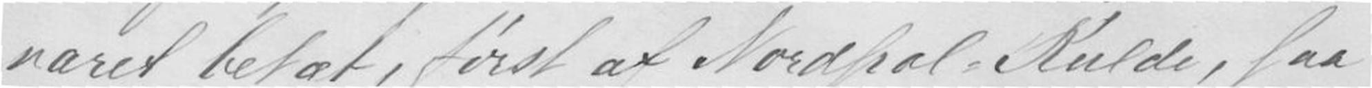næret betæt, først at Nordpol=Kulde, faa
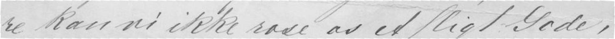re kan vi ikke rose os et sligt Møde,
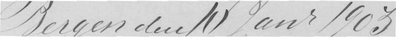Bergen den 10 Janr 1903
